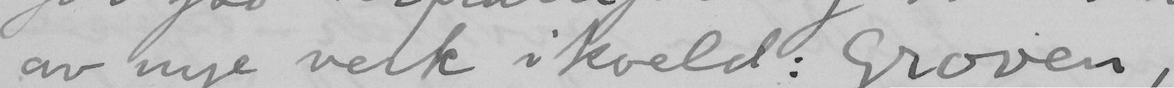av nye verk ikveld: Groven,
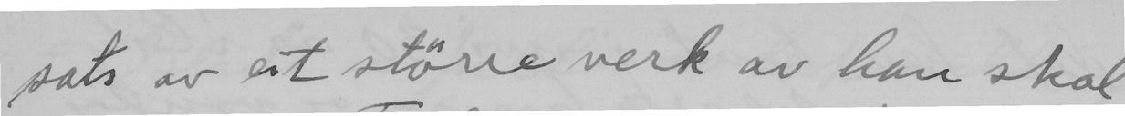sats av eit större verk av han skal
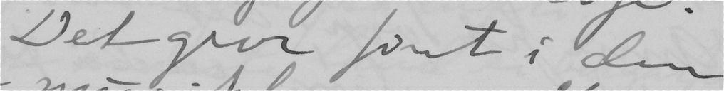Det gror fint i den
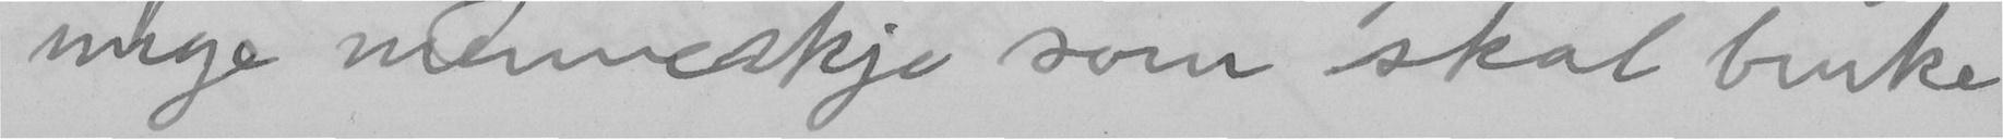unge menneskje som skal bruke
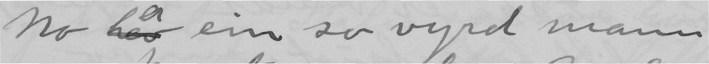No er ein so vyrd mann
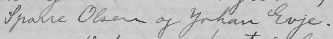Sparre Olsen og Johan Evje.
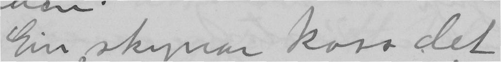Ein skyner koss det
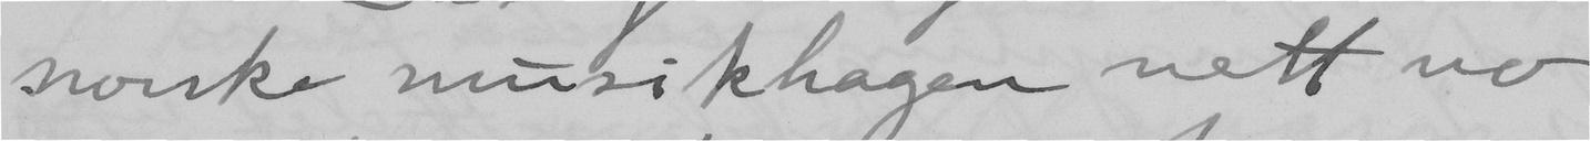norske musikhagen nett no.
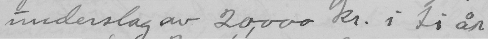underslag av 20,000 kr. i ti år.
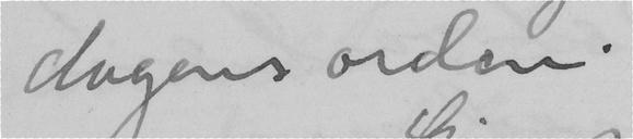dagens orden.
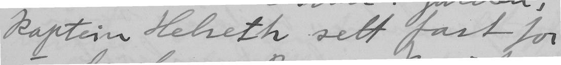Kaptein Helseth sett fast for
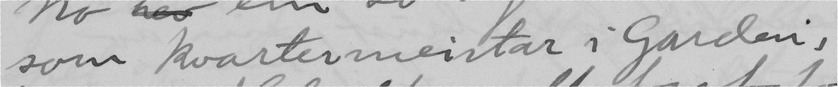som kvartermeistar i Garden.
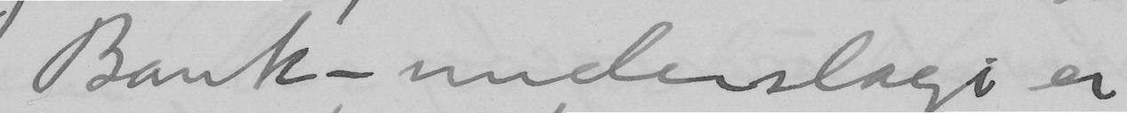Bank-underslagi er
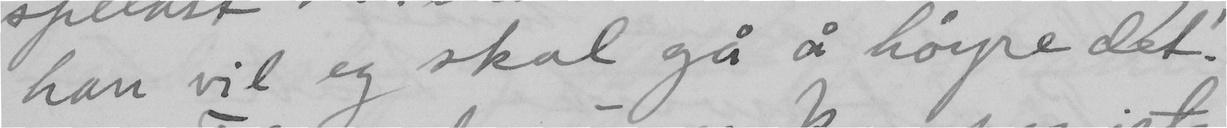han vil eg skal gå å höyre det.
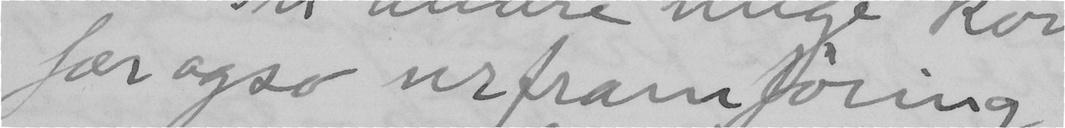fær ogso urframföring
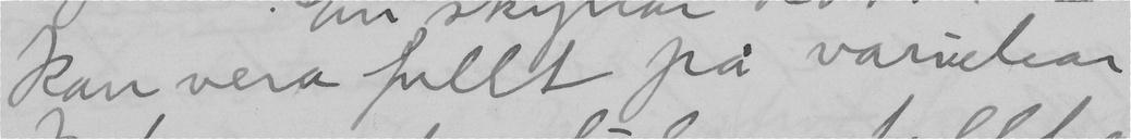kan vera fullt på varietear
